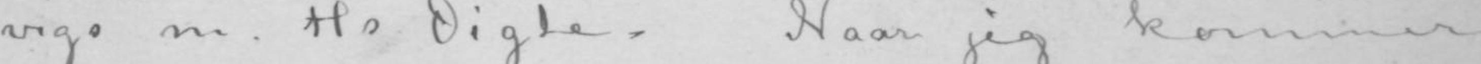vigs m. Fls Digte. Naar jeg kommer
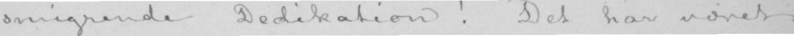smigrende Dedikation! Det har været
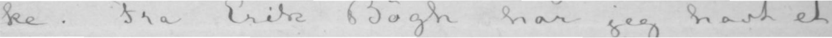ke. Fra Erik Bøgh har jeg havt et
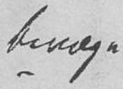bevæge-
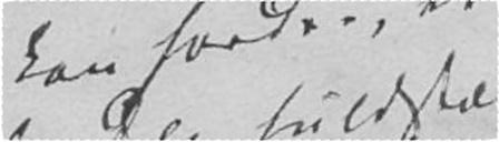kan fordre, at
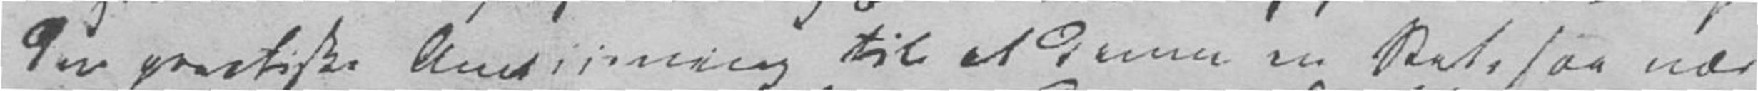den practiske Anviisning til at danne en Stat, saa nær
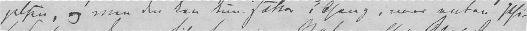gelsen, og men den kan kun sættes i Gang, naar enten Phi-
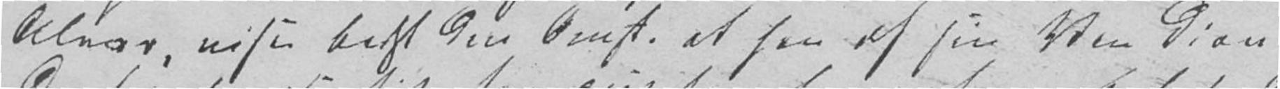Alvor, viser best den Omst. at han af sin Ven Dion
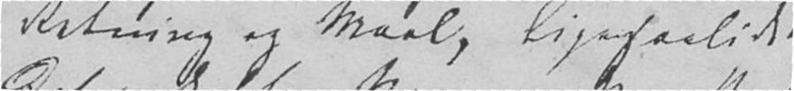Retning og Maal, ligesaalidt
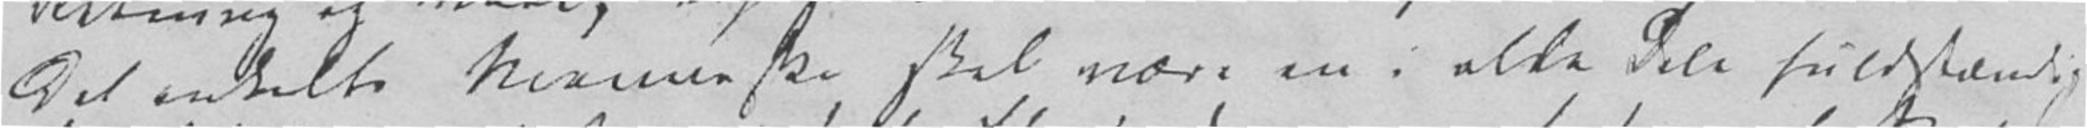det enkelte Menneske skal være en i alle Dele fuldstændig
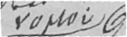Nomoi
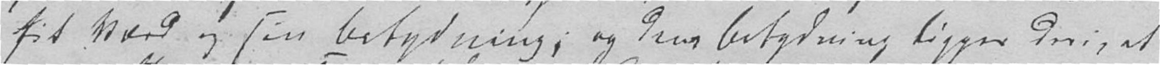sit Værd og sin Betydning, og dens Betydning ligger deri, at
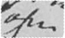som
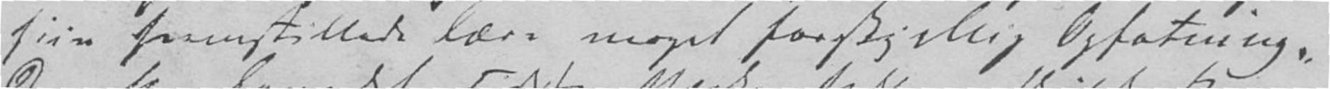hiin fremstillede Lære meget forskjellig Opfatning,
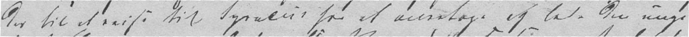des til at reise til Syracus for at overtage og lede den unge
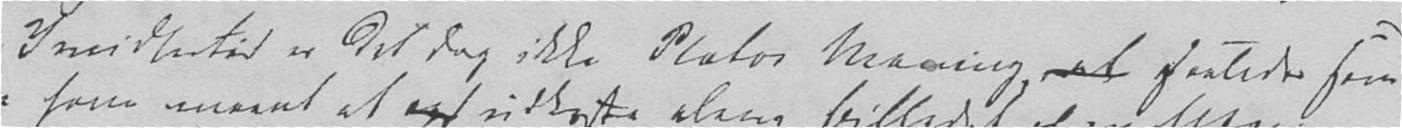Imidlertid er det dog ikke Platos Mening, at saaledes som
